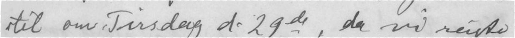til om Tirsdag d. 29de, da vi reiste
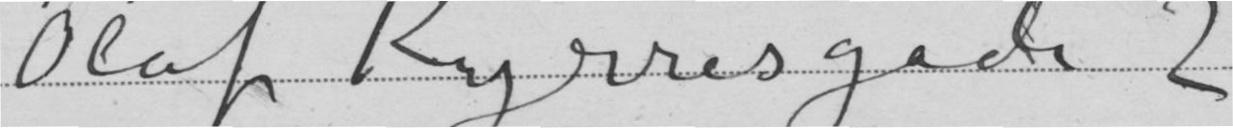Olaf Kyrresgade 2
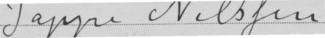Jappe Nilssen
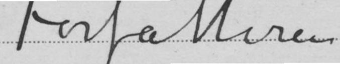Forfatteren
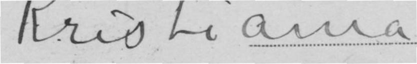Kristiania
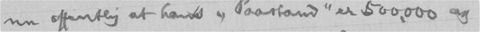nu offentlig at hans "Paastand" er 500.000 og
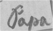Papa
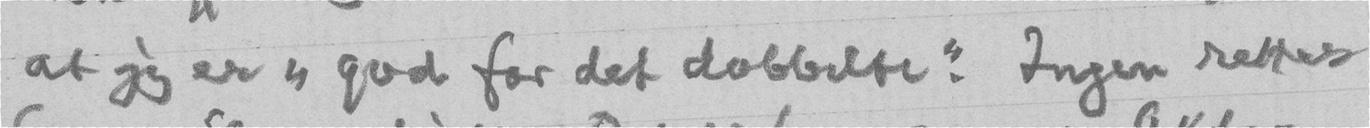at jeg er "god for det dobbelte". Ingen retter
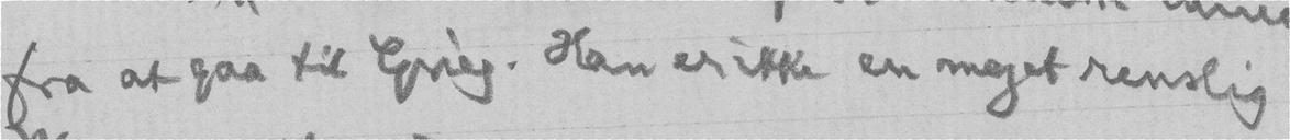fra at gaa til Grieg. Han er ikke en meget renslig
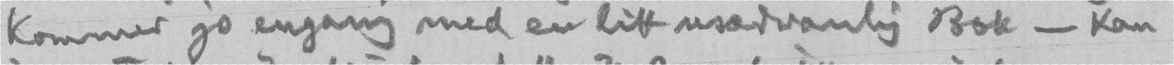kommer jo engang med en litt usædvanlig Bok - kan
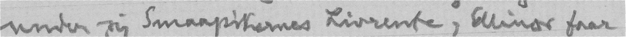under sig Smaapikernes Livrente, Ellinor faar
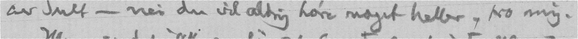av Sult - nei du vil aldrig høre noget heller, tro mig.
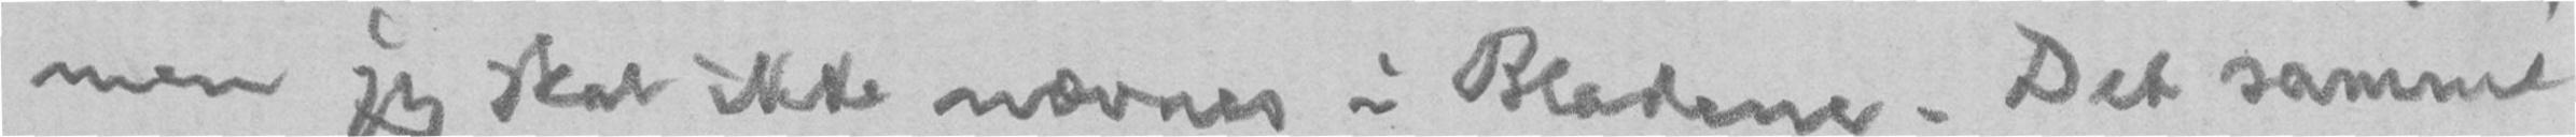men jeg skal ikke nævnes i Bladene. Det samme
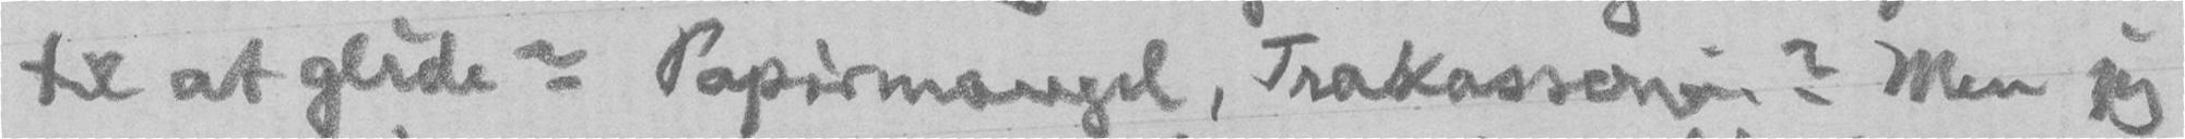til at glide? Papirmangel, Trakasserier? Men jeg
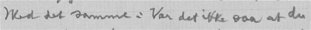Med det samme: Var det ikke saa at du
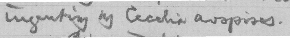ingenting og Cecilia avspises.
